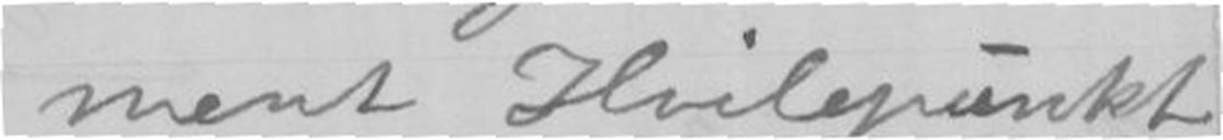ment Hvilepunkt
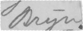Bryr
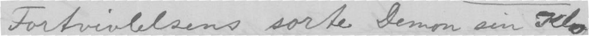Fortvivlelsens sorte Demon sin Klo
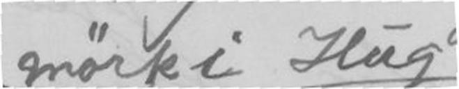mørk i Hug,
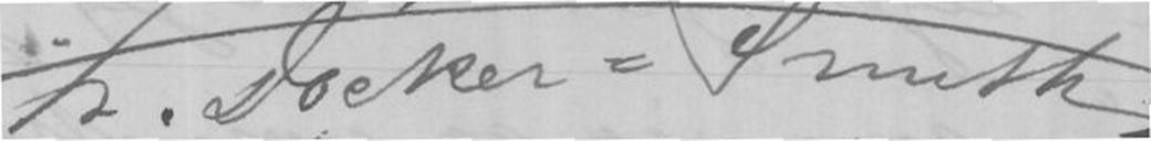Fr. Döcker=Smith
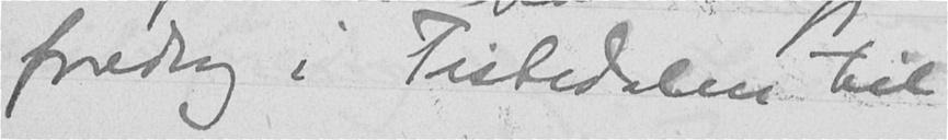foredrag i Tistedalen til
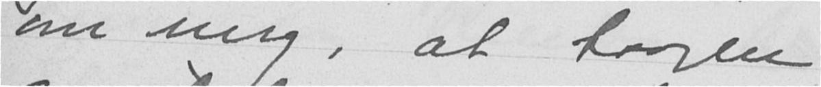om mrg, at taagen
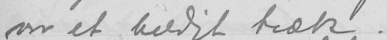var et heldigt træk.
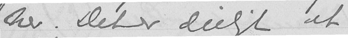her. Det er deiligt at
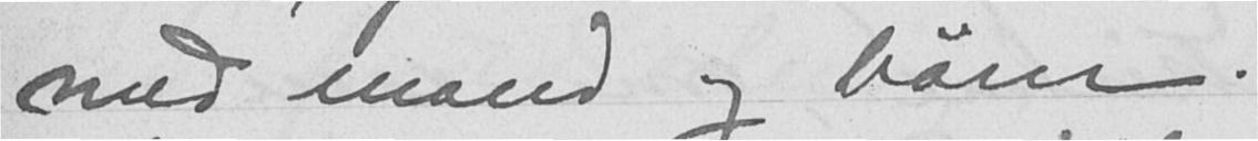med mand og børn.
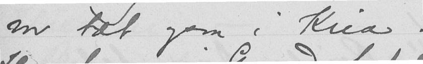var tæt ogsaa i Kria.
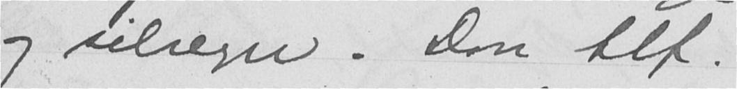og silregn. Don tlf.
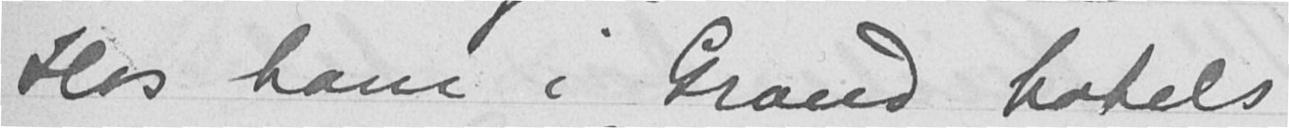Hos ham i Grand hotels
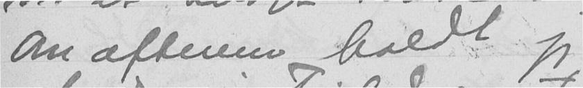Om aftenen holdt jeg
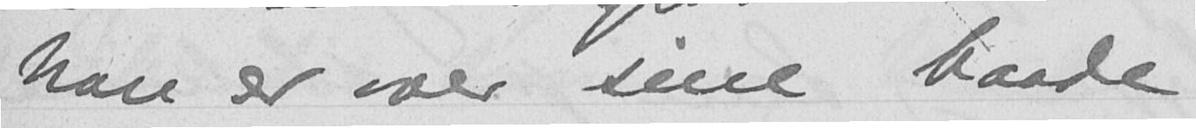han er over sine baade
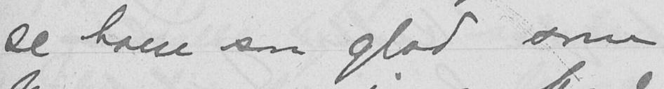se ham saa glad som
